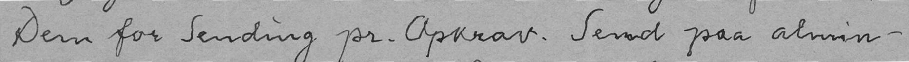Dem for Sending pr. Opkrav. Send paa almin-
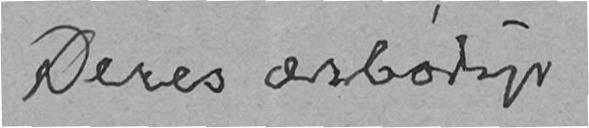Deres ærbødige
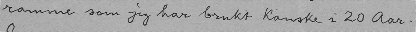ramme som jeg har brukt kanske i 20 Aar.
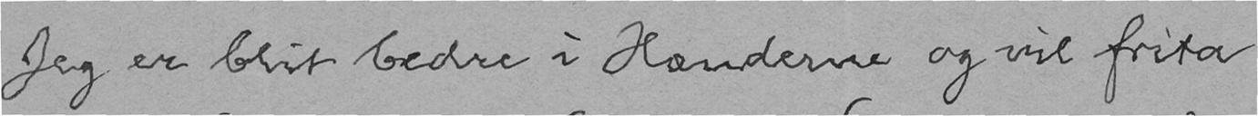Jeg er blit bedre i Hænderne og vil frita
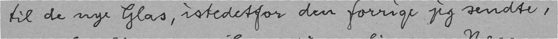til de nye Glas, istedetfor den forrige jeg sendte,
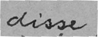disse
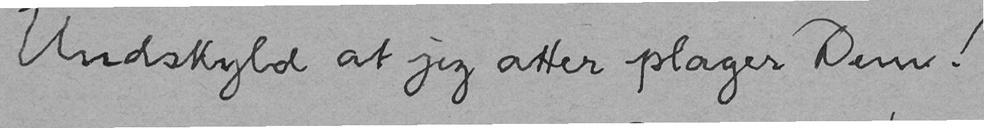Undskyld at jeg atter plager Dem!
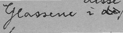Glassene i de
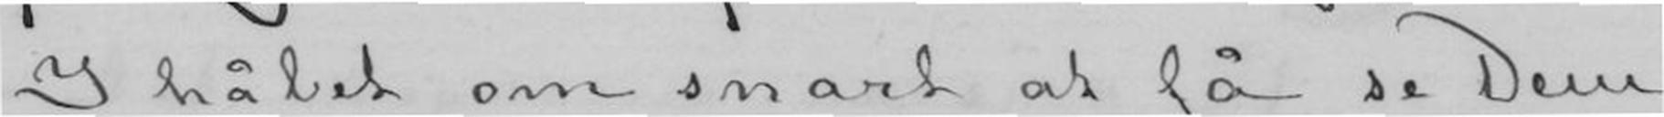I håbet om snart at få se Dem
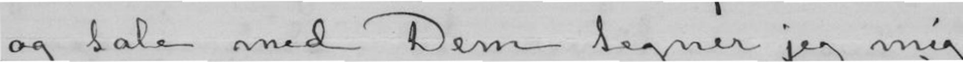og tale med Dem tegner jeg mig
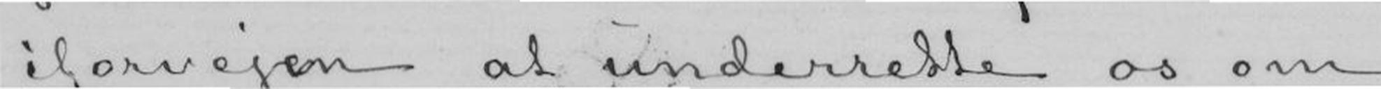iforvejen at underrette os om
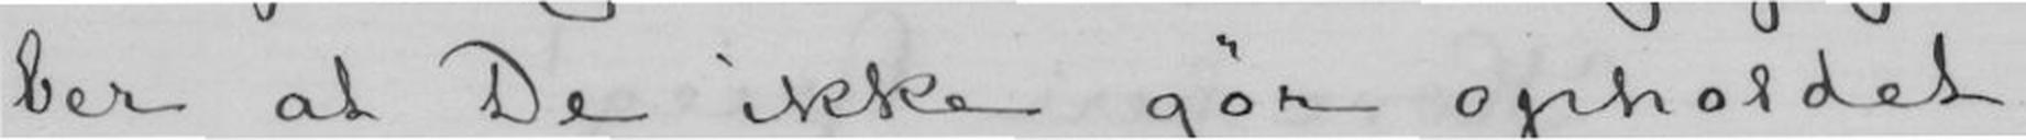ber at De ikke gør opholdet
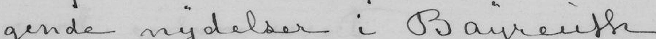gende nydelser i Bayreuth
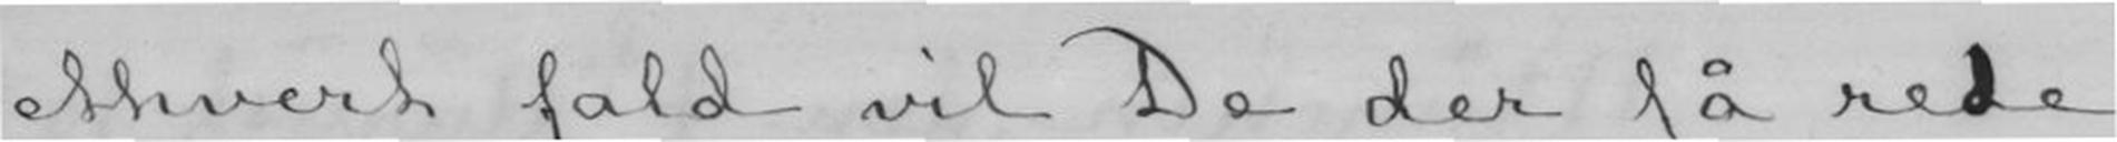ethvert fald vil De der få rede
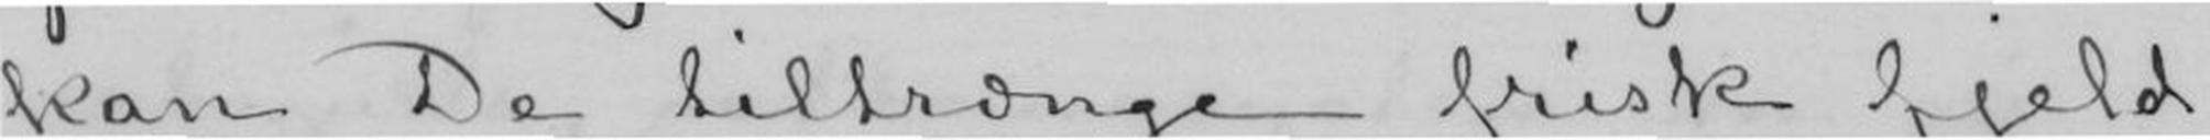kan De tiltrænge frisk fjeld-
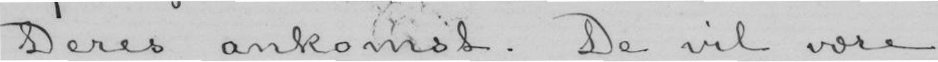Deres ankomst. De vil være
